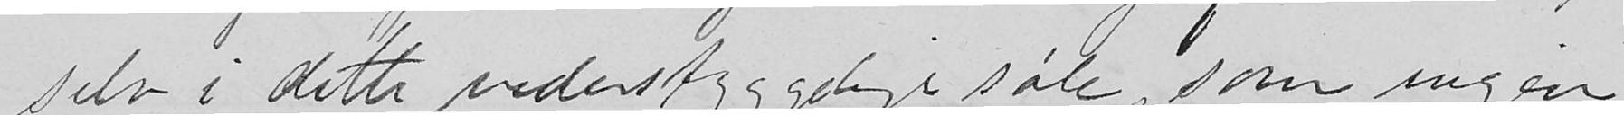selv i dette vederstyggelige søle, som ingen
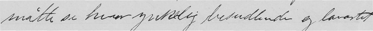måtte se hvor ynkelig besudlende og lavartet
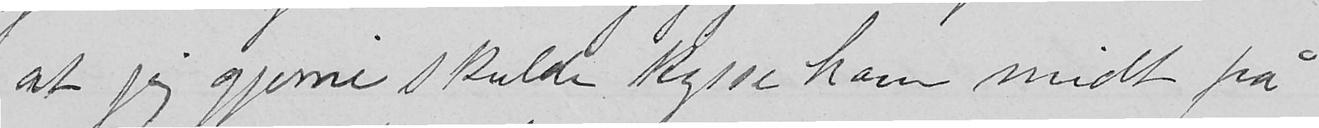at jeg gjerne skulde kysse ham midt på
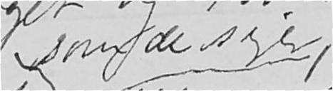som de siger,
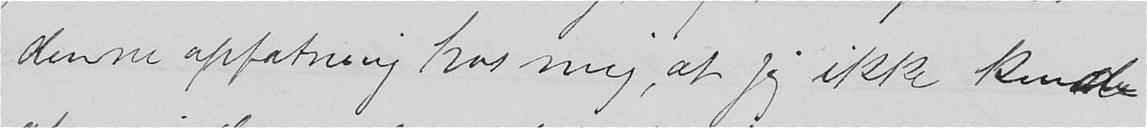denne opfatning hos mig, at jeg ikke kunde
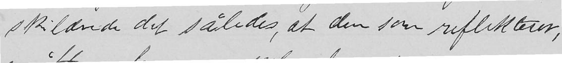skildrede det således, at den som reflekterer,
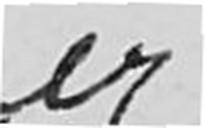er
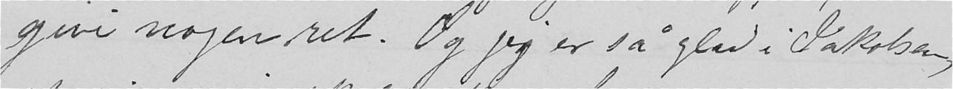give nogen ret. Og jeg er så glad i Jakobsen,
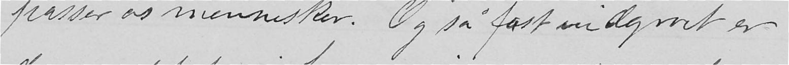passer os mennesker. Og så fast indgroet er
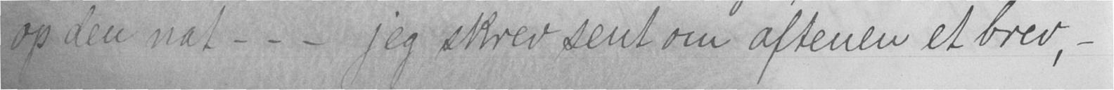op den nat - - - jeg skrev sent om aftenen et brev, -
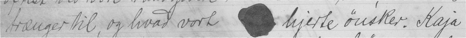trænger til, og hvad vort hjerte ønsker. Kaja
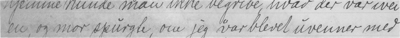en og mor spurgte, om jeg var blevet uvenner med
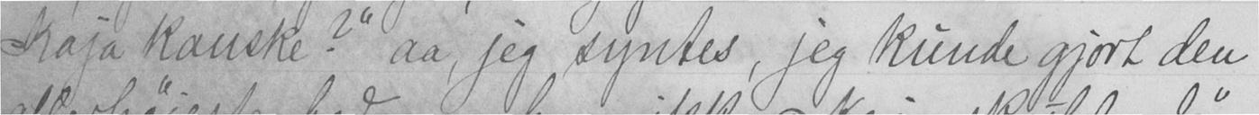Kaja kanske? aa, jeg syntes, jeg kunde gjørt den
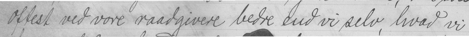oftest ved vore raadgivere bedre end vi selv, hvad vi
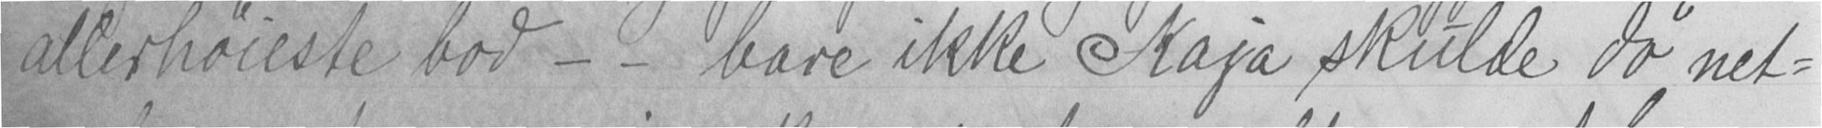allerhøieste bod - - bare ikke Kaja skulde dø net-
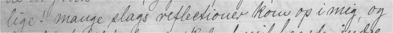lige! mange slags reflectioner kom op i mig, og
